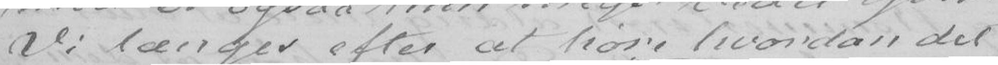Vi længes efter at høre hvodan det
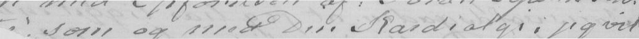" som og med Din Kardialgi; jeg vil
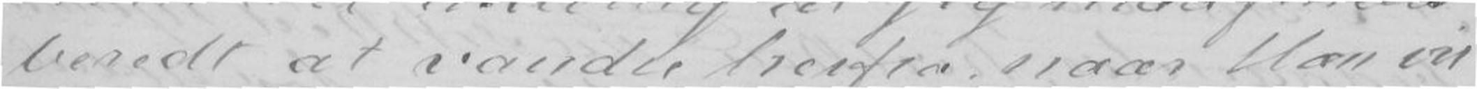beredt at vandre herfra naar Han vil
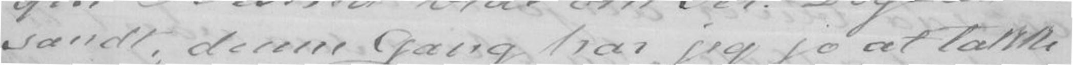sandt, denne Gang har jeg jo at takke
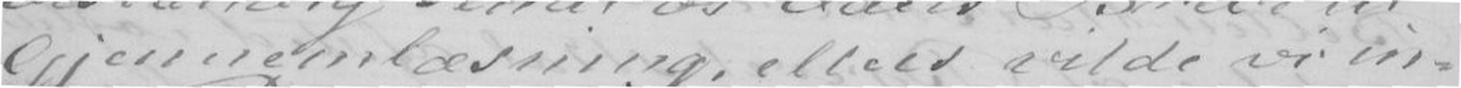Gjennemlæsning, ellers vilde vi in-
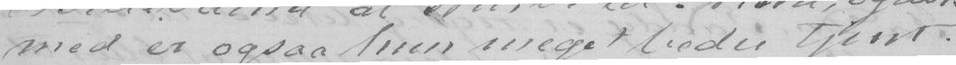med er ogsaa hun meget bedre Tjent.
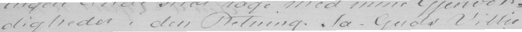digheder i den Retning. Ja - Guds Villie
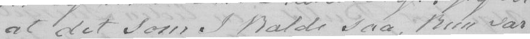at det som I kalde saa, kun var
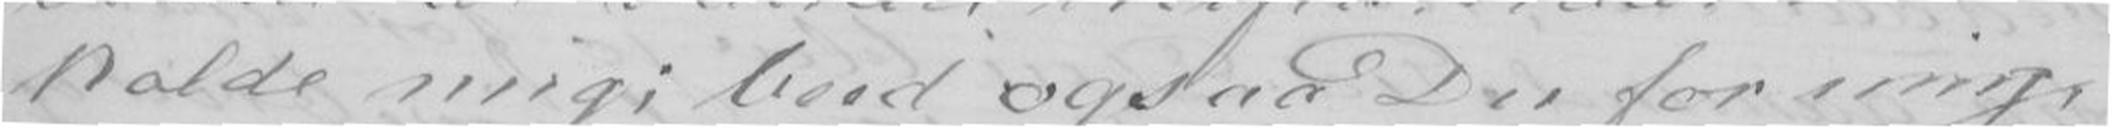kalde mig; beed ogsaa Du for mig,
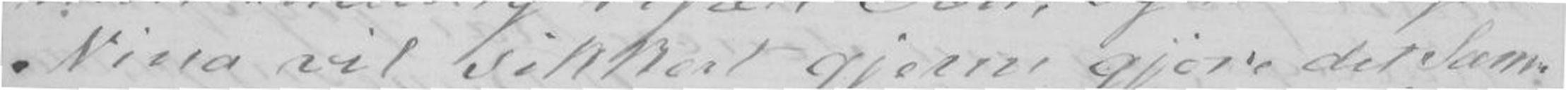Nina vil sikkert gjerne gjøre det Sam-
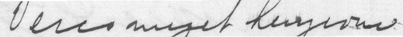Deres meget hengivne
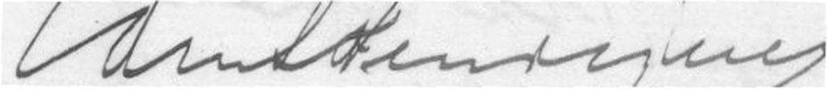Edm. Henriques.
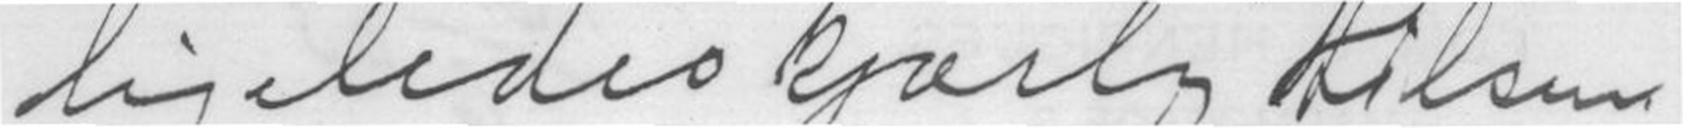ligeledes kjærlig Hilsen
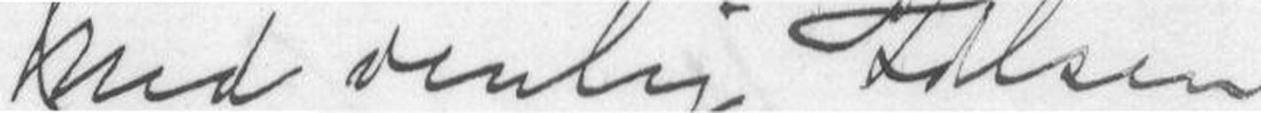med venlig hilsen
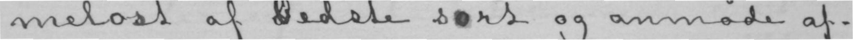melost af dbedste sort og anmode af-
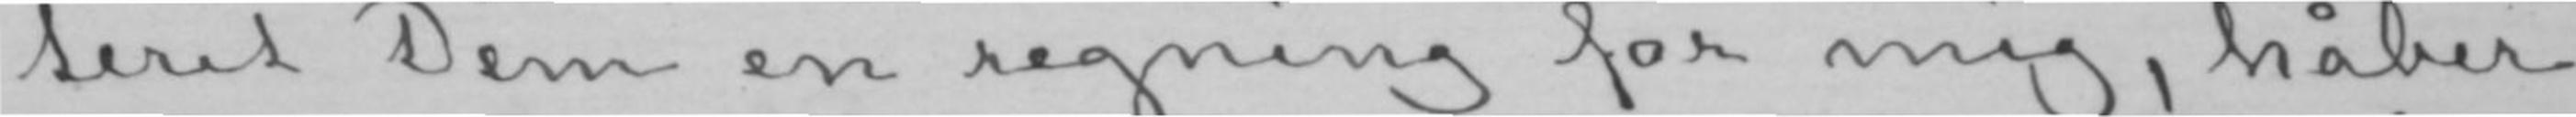teret Dem en regning for mig, håber
